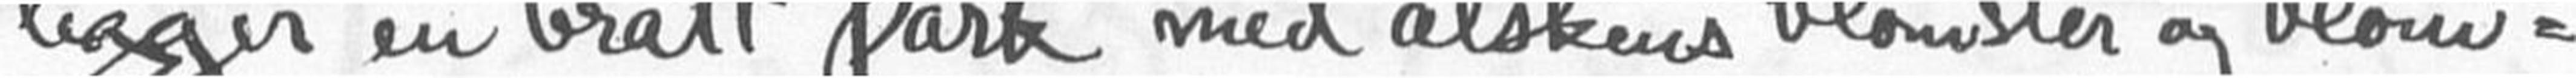ligger en bratt park med alskens blomster og blom-
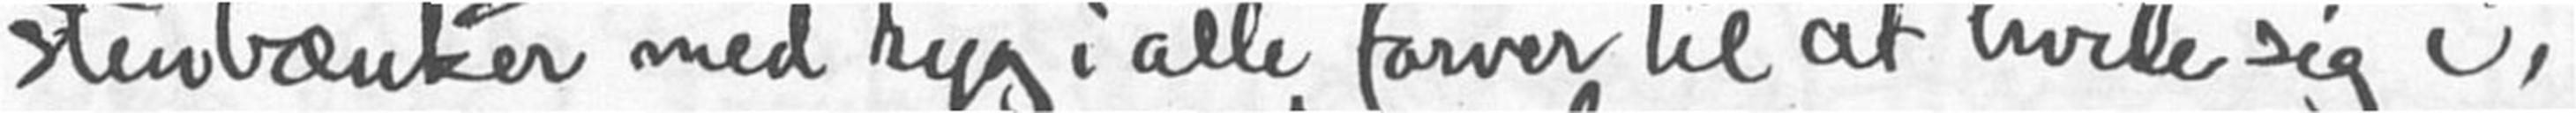stenbænker med ryg i alle farver til at hvile sig i,
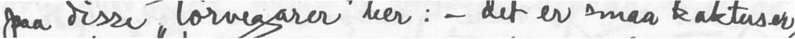paa disse "torvegarer" her: - det er smaa kaktuser
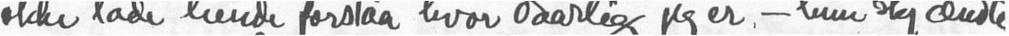ikke lade hende forstaa hvor daarlig jeg er - hun skjændte
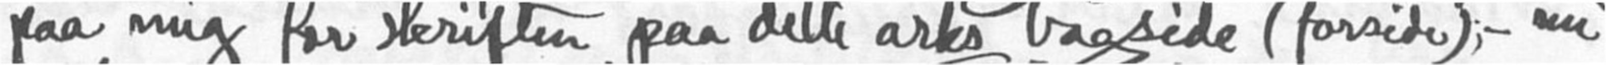paa mig for skriften paa dette arks bagside (forside); - nu
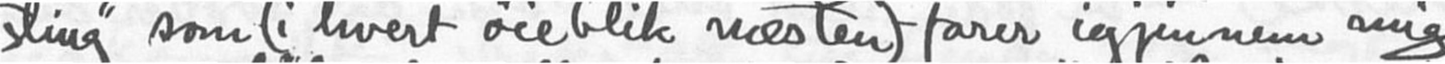"sting" som (i hvert øieblik næsten) farer igjennem mig
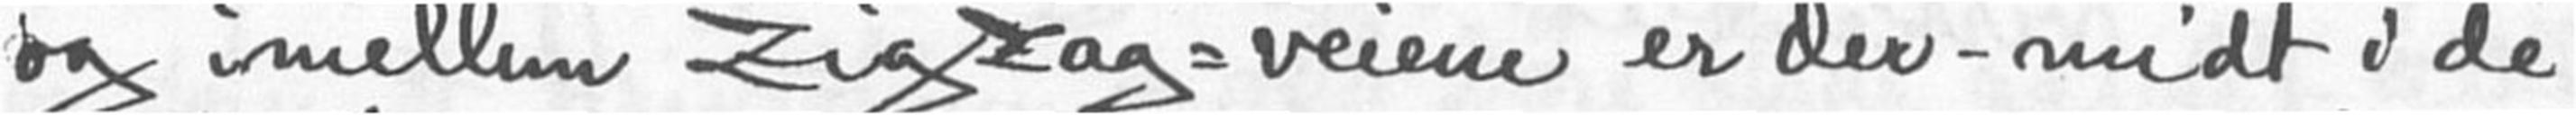og imellem zigzag-veiene er der - midt i de
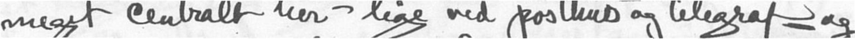meget centralt her - lige ved posthus og telegraf- og
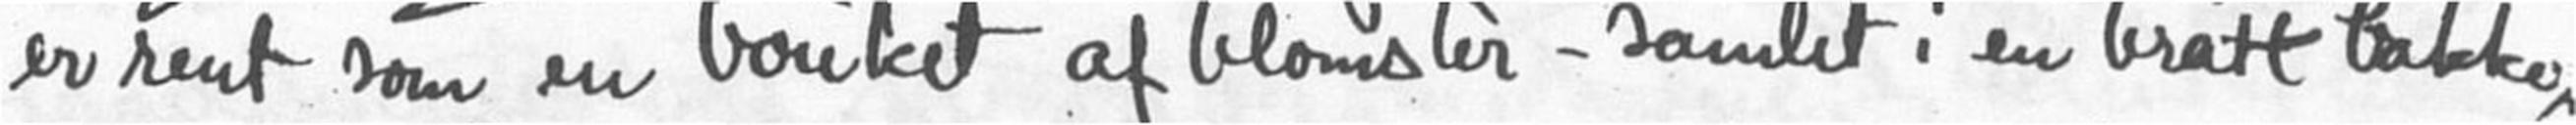er rent som en bouket af blomster - samlet i en bratt bakke,
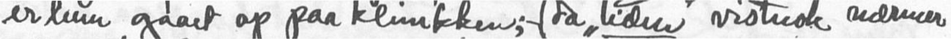er hun gaaet op paa klinikken;- (da "tiden" vistnok nærmer
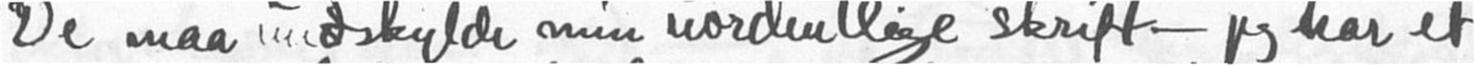De maa undskylde min uordentlige skrift - jeg har et
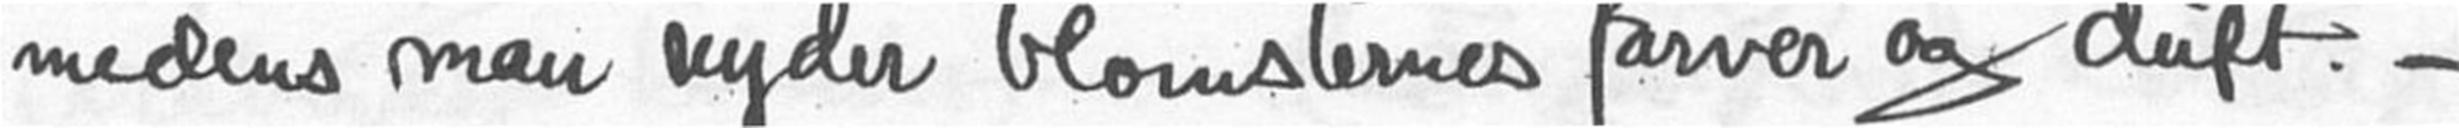medens man nyder blomsternes farver og duft. -
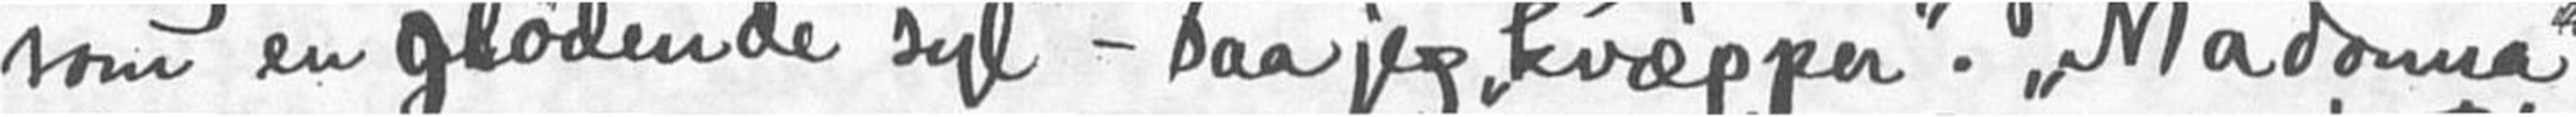som en glødende syl - saa jeg "kvæpper". "Madonna"
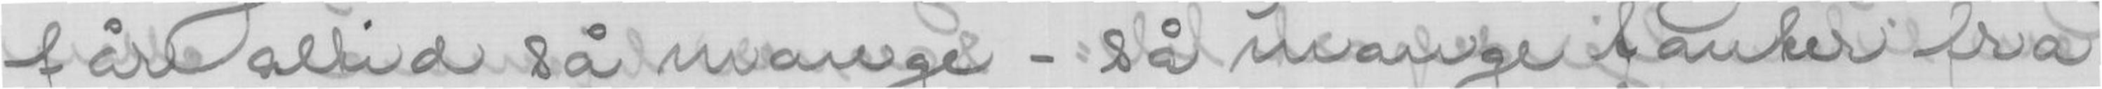får altid så mange - så mange tanker fra
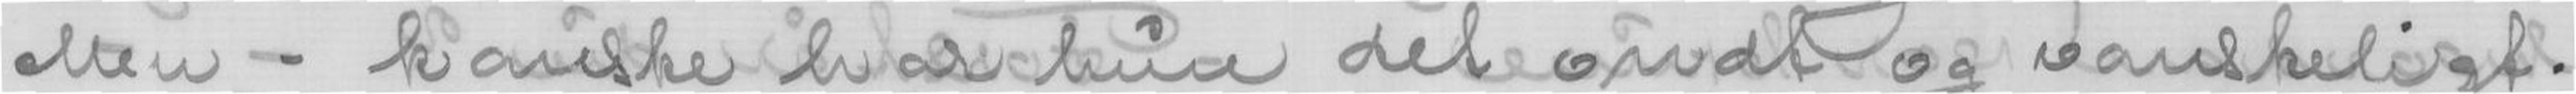Men - kanske har hun det ondt og vanskeligt.
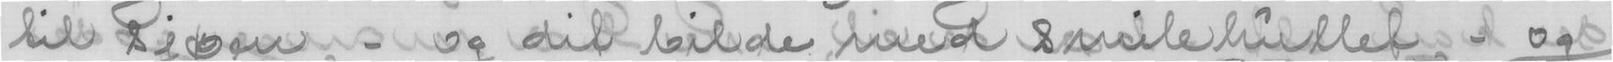til sjøen, - og dit bilde med smilehullet, - og
